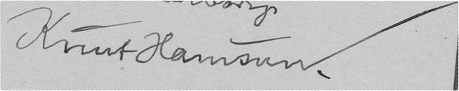Knut Hamsun.
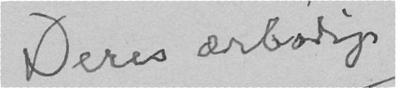Deres ærbødige
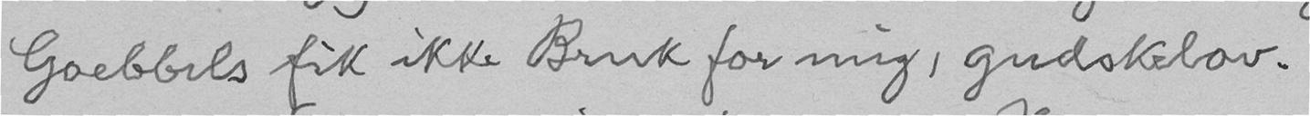Goebbels fik ikke Bruk for mig, gudskelov.
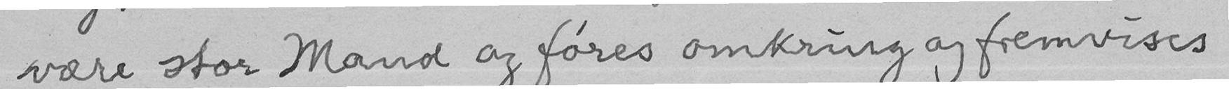være stor Mand og føres omkring og fremvises
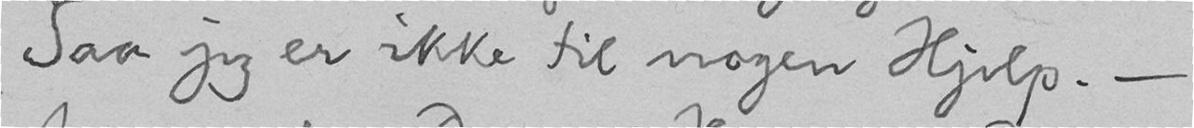Saa jeg er ikke til nogen Hjelp. -
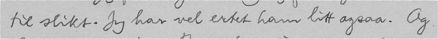til slikt. Jeg har vel ertet ham litt ogsaa. Og
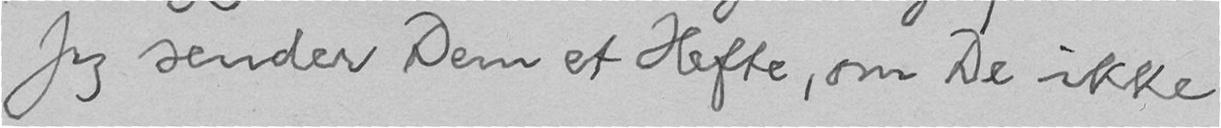Jeg sender Dem et Hefte, om De ikke
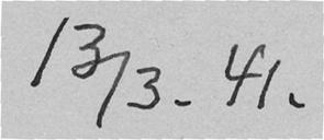13/3. 41.
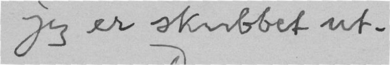jeg er skubbet ut.
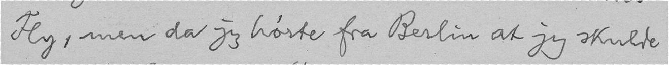Fly, men da jeg hørte fra Berlin at jeg skulde
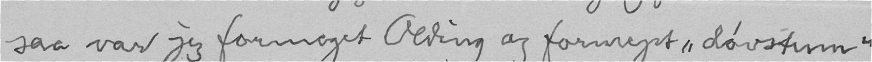saa var jeg formeget Olding og formeget "døvstum"
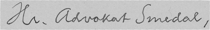Hr. Advokat Smedal,
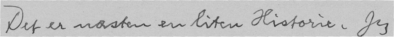Det er næsten en liten Historie. Jeg
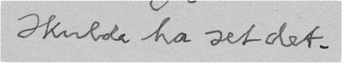skulde ha set det.
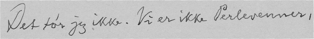Det tør jeg ikke. Vi er ikke Perlevenner,
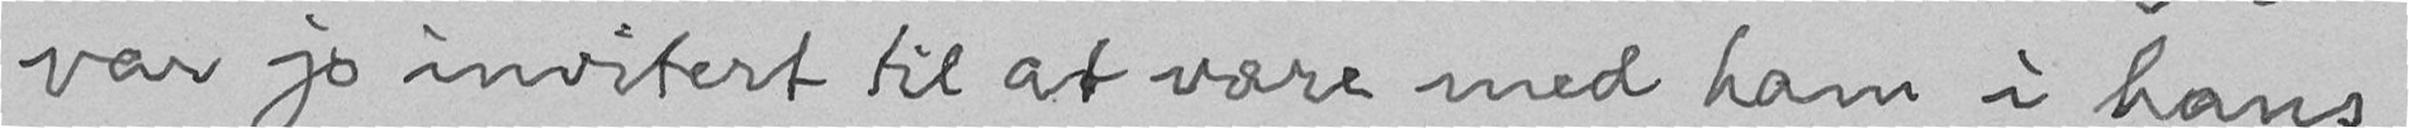var jo invitert til at være med ham i hans
